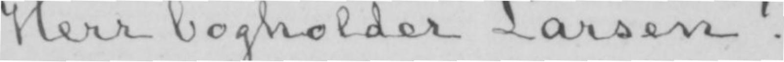Herr bogholder Larsen!
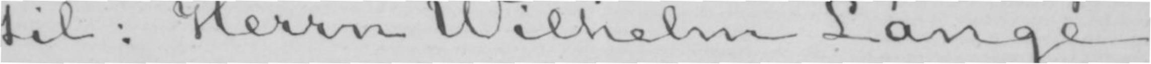til: Herrn Wilhelm Lange.
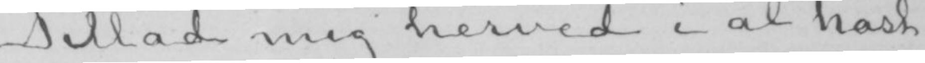Tillad mig herved i al hast
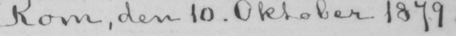Rom, den 10. Oktober 1879.
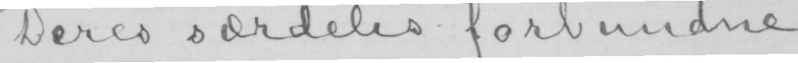Deres særdeles forbundne
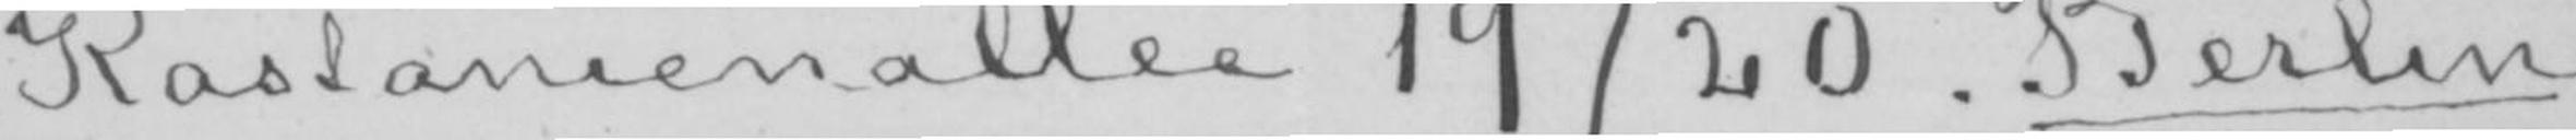Kastanienallee 19/20. Berlin.
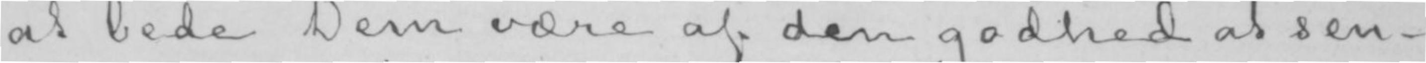at bede Dem være af den godhed at sen-
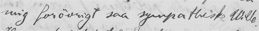mig forøvrigt saa sympathiske Wille.
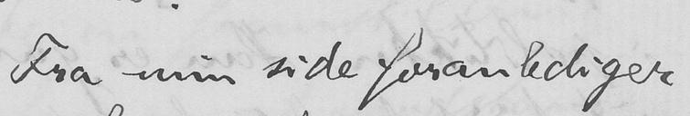Fra min side foranlediger
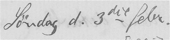Søndag d. 3die febr.
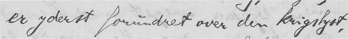er yderst forundret over den krigslyst,
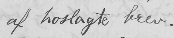af hoslagte brev.
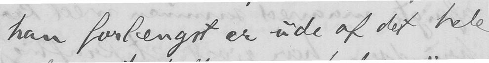han forlængst er ude af det hele
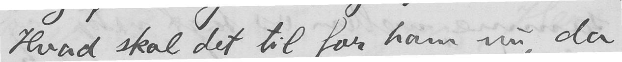Hvad skal det til for ham nu, da
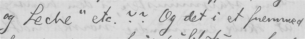og Leche" etc.?? Og det i et fremmed
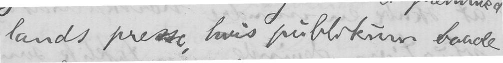lands presse, hvis publikum baade
